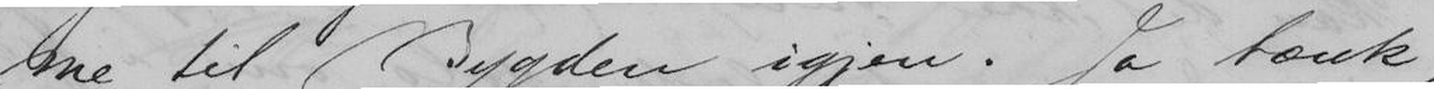me til Bygden igjen. Ja tænk,
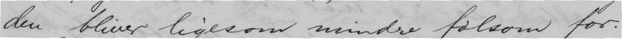den bliver ligesom mindre følsom for.
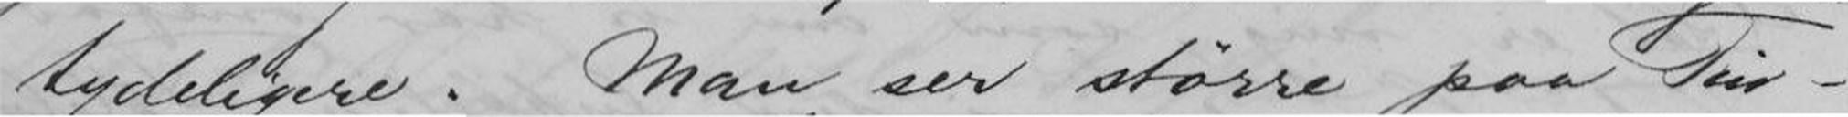tydeligere. Man ser større paa Tin-
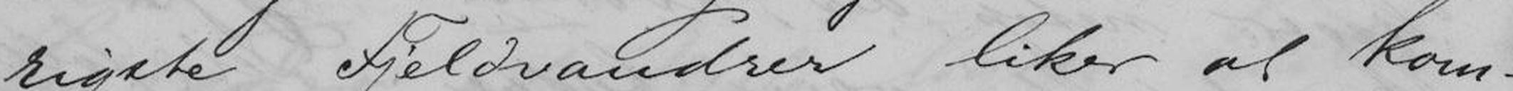rigste Fjeldvandrer liker at kom-
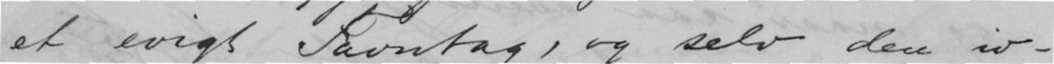et evigt Favntag, og selv den iv-
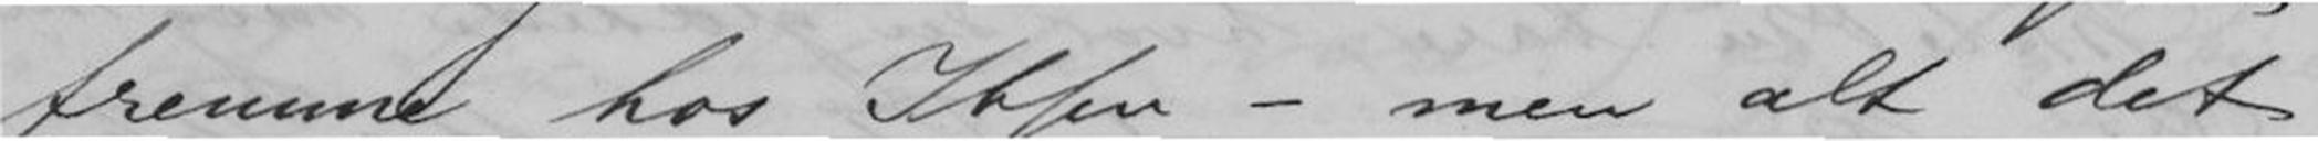fremme hos Ibsen - men alt det
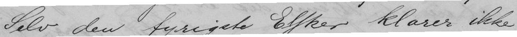Selv den fyrigste Elsker klarer ikke
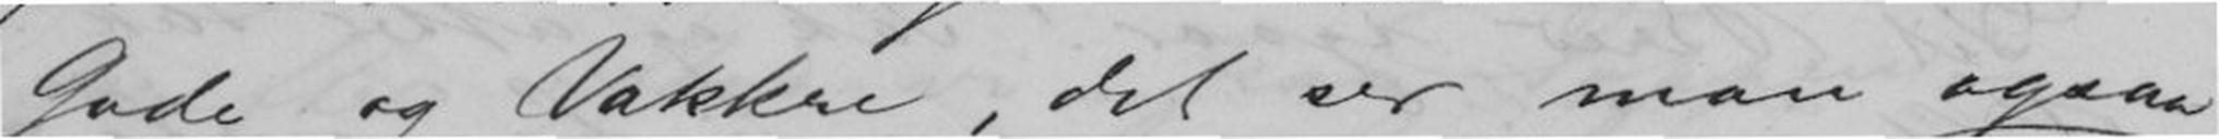Gode og Vakkre, det ser man ogsaa
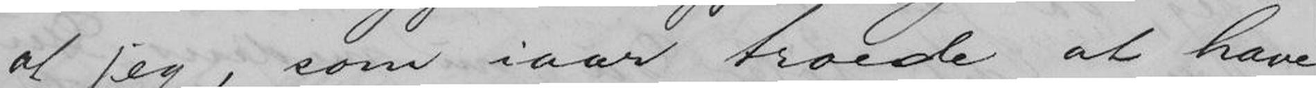at jeg, som iaar troede at have
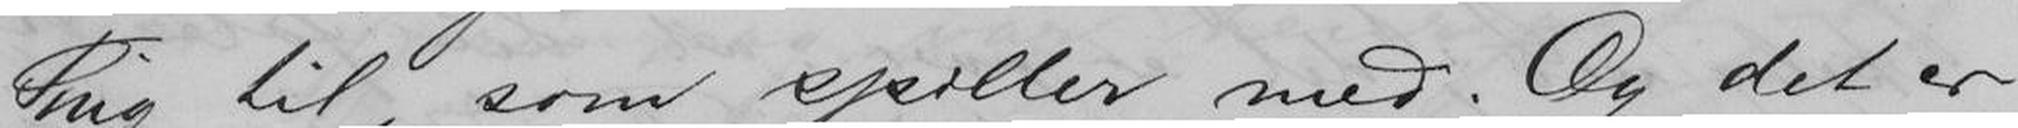Ting til, som spiller med. Og det er
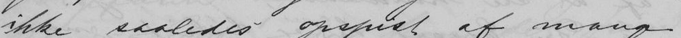ikke saaledes opspist af mange
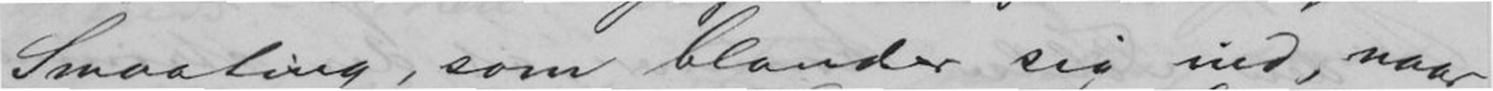Smaating, som blander sig ind, naar
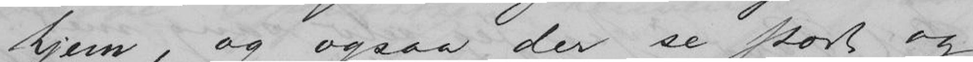hjem, og ogsaa der se stort og
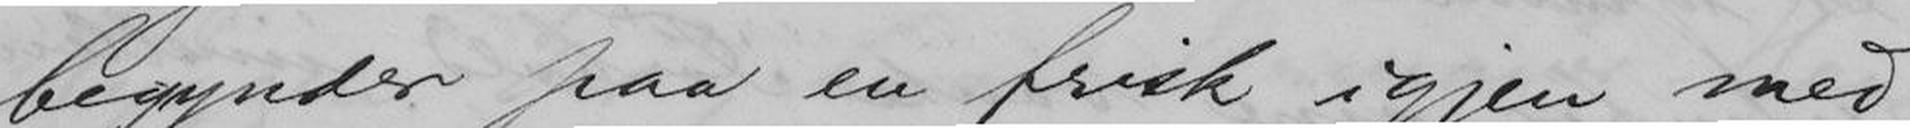begynder paa en frisk igjen med
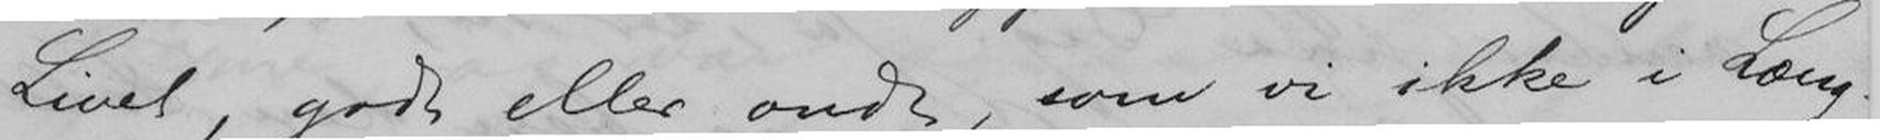Livet, godt eller ondt, som vi ikke i Læng-
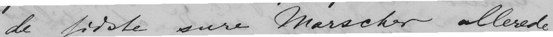de sidste sure Marscher allerede
